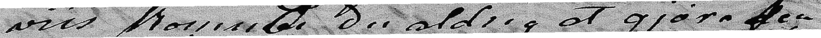viis kommer Du aldrig at gjøre den
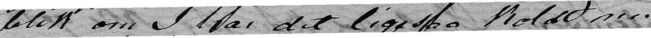blik om I har det ligesaa koldt nu
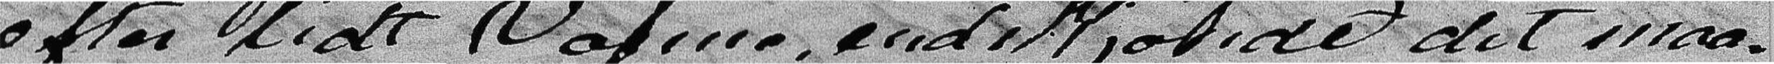efter lidt Varme, endskjøndt det maa-
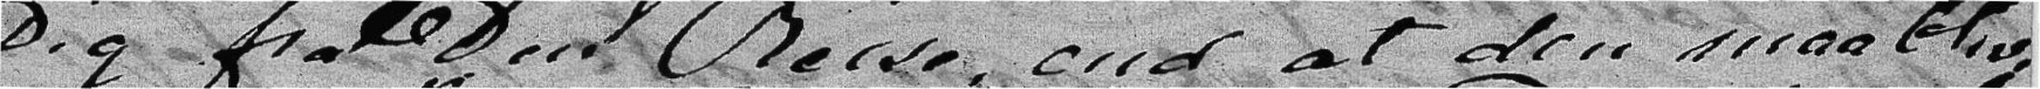Dig fra Din Reise, end at den maa blive
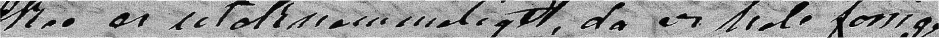skee er utaknemmeligt, da vi hele forrige
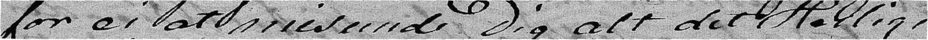for ei at misunde Dig alt det Herlige
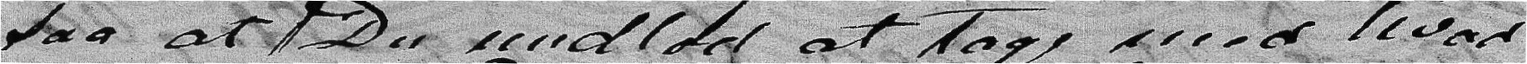saa at Du undlod at tage med hvad
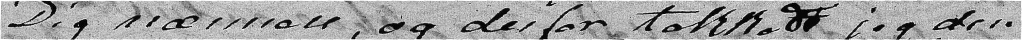Dig nærmere, og derfor takkede jeg den
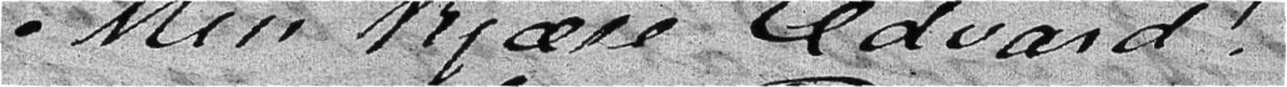Min kjære Edvard!
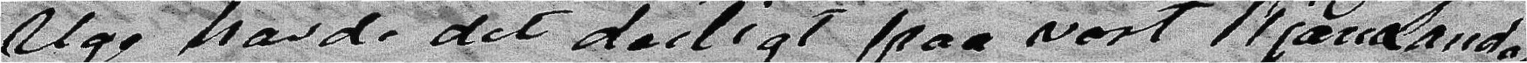Uge havde det deiligt paa vort kjære Landaas.
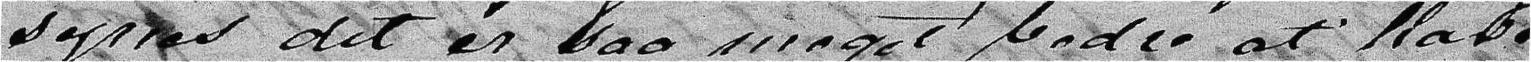synes det er saa meget bedre at have
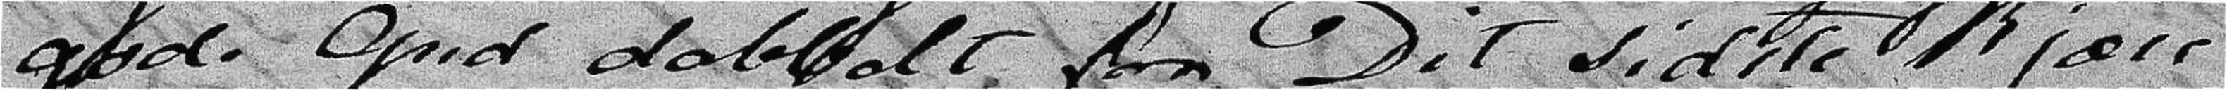gode Gud dobbelt for Dit sidste kjære
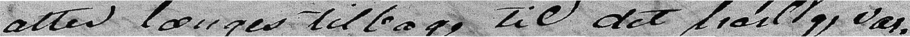atter længes tilbage til det herlige var-
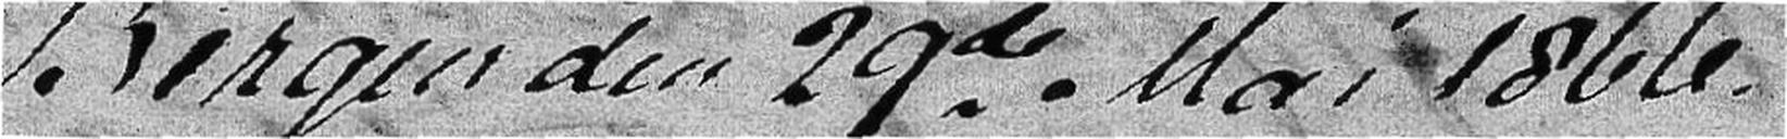Bergen den 29de Mai 1866.
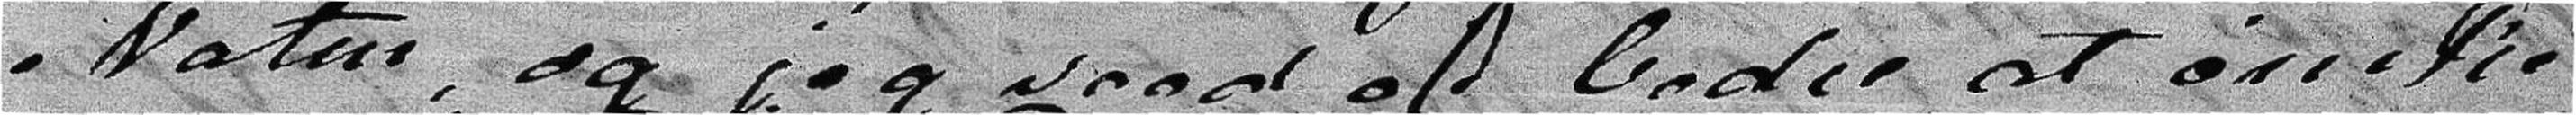Natur, og jeg veed ei bedre at ønske
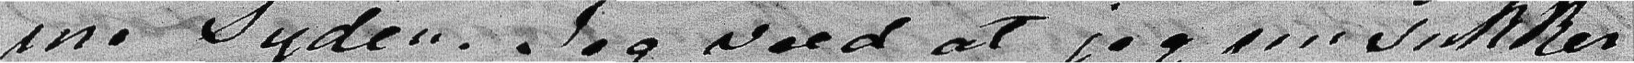me Syden. Jeg veed at jeg nu sukker
